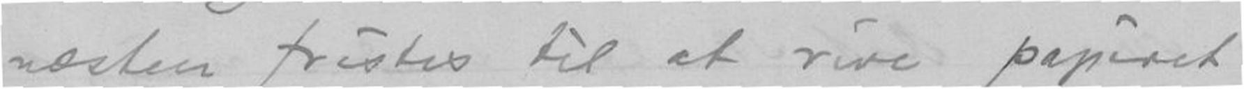næsten fristes til at rive papiret
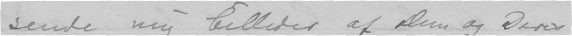sende mig billeder af Dem og Deres
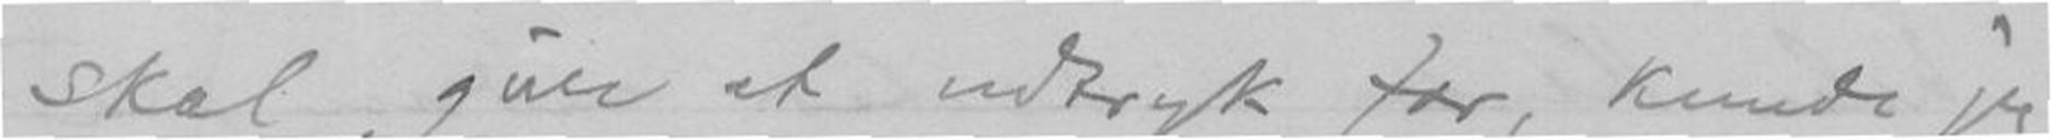skal give et udtryk for, kunde jeg
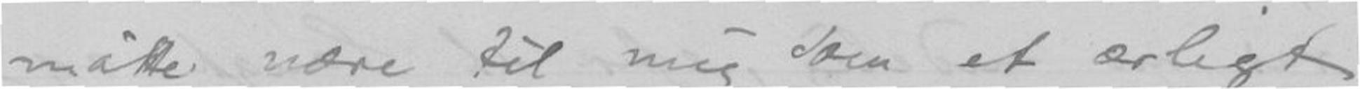måtte nære til mig som et ærligt
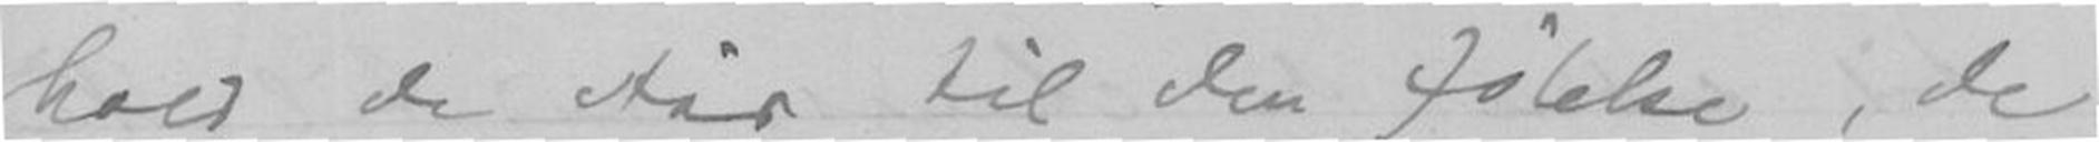hold de står til den følelse, de
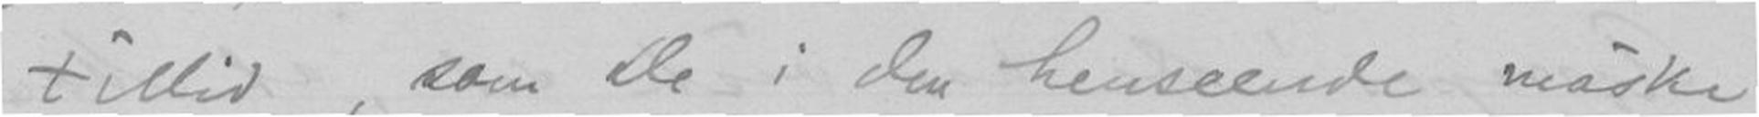tillid, som De i den henseende måske
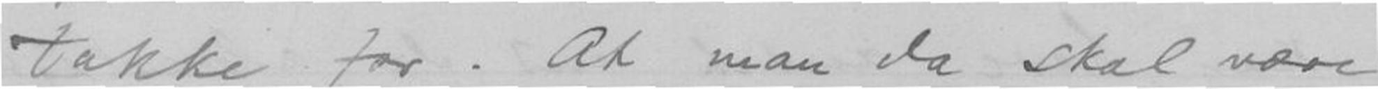takke for. At man da skal være
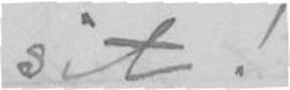sit!
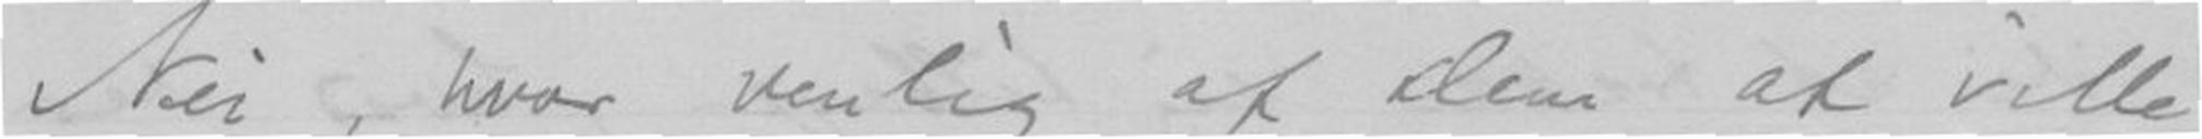Nei, hvor venlig af Dem at ville
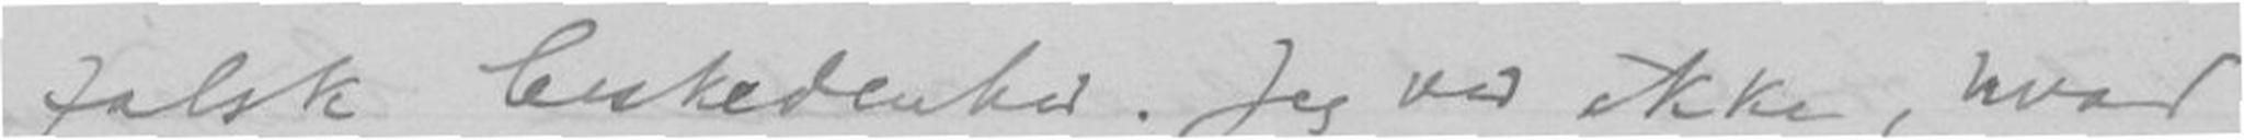falsk beskedenhed. Jeg ved ikke, hvad
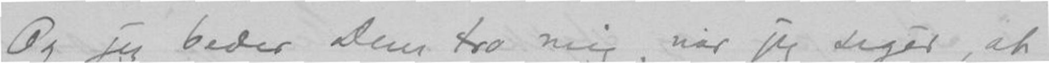Og jeg beder Dem tro mig, når jeg siger, at
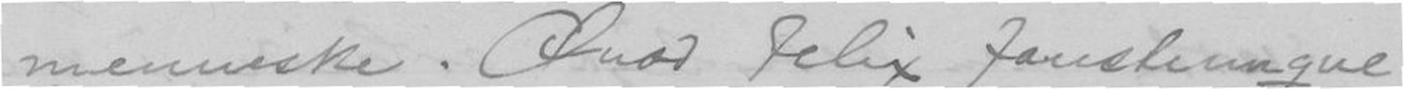menneske. Quad felix fansteunque
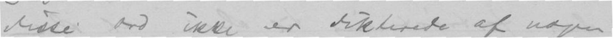disse ord ikke er dikterede af nogen
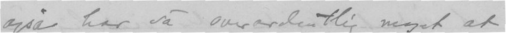også har så overordentlig meget at
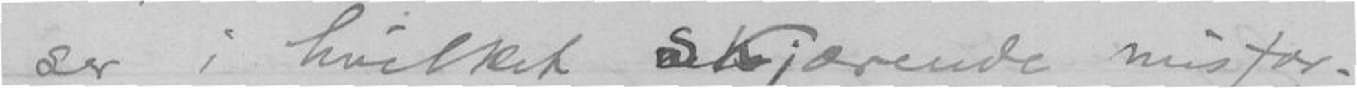ser i hvilket skjærende misfor-
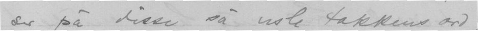ser på disse så usle takkens ord,
